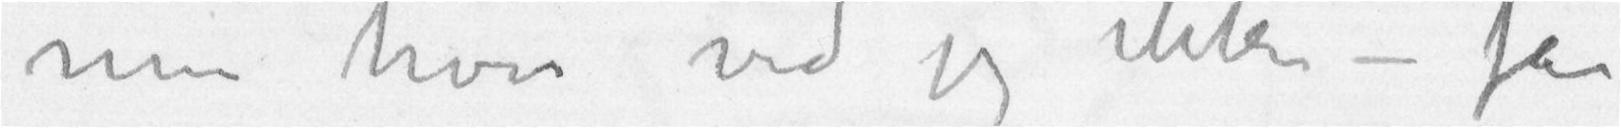menhvor ved jeg ikke – for
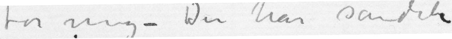for mig – Du har sandeli
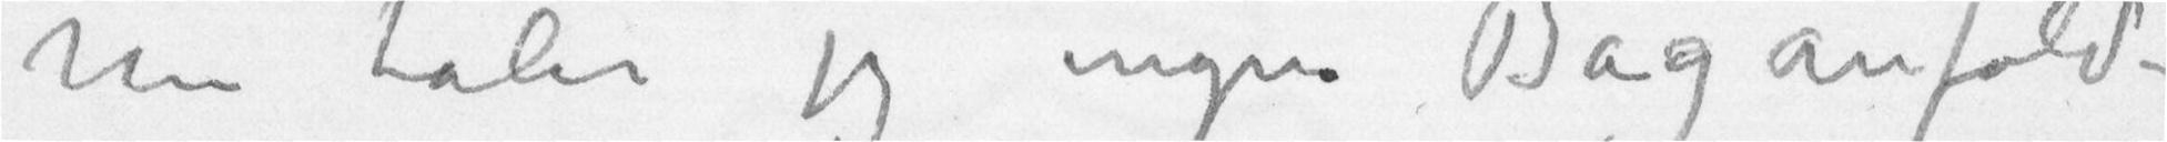nu tåler jeg ingen Baganfald –
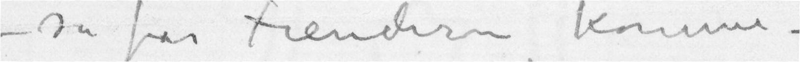– så får Fienderne komme –
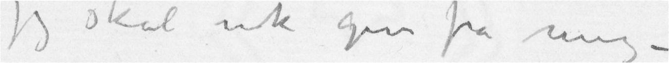jeg skal nok give fra mig –
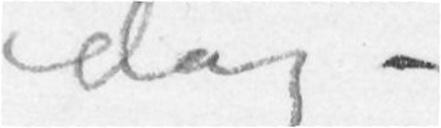idag –
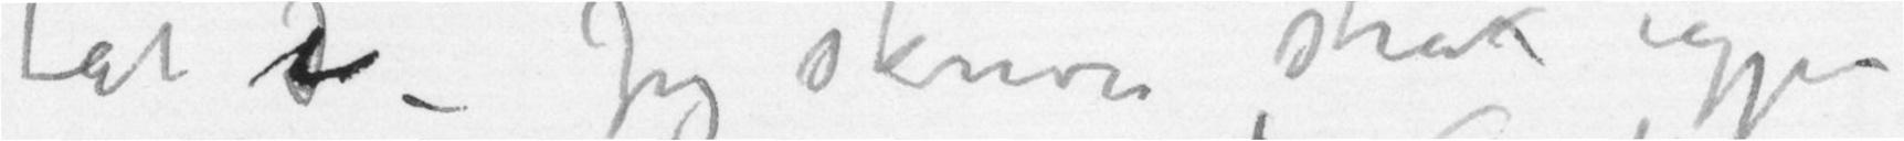tat di – Jeg skriver strax igjen
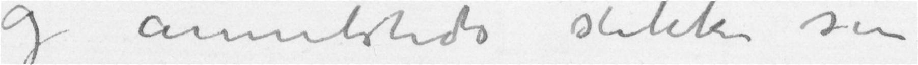og annetsteds stikke sine
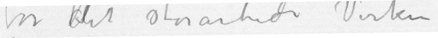for Dit storartede Virken
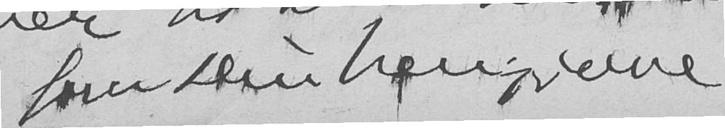fra Din hengivne
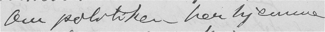Om politiken her hjemme
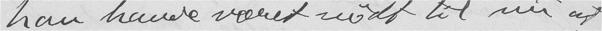han havde været nødt til nu at
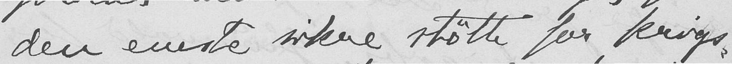den eneste sikre støtte for krigs-
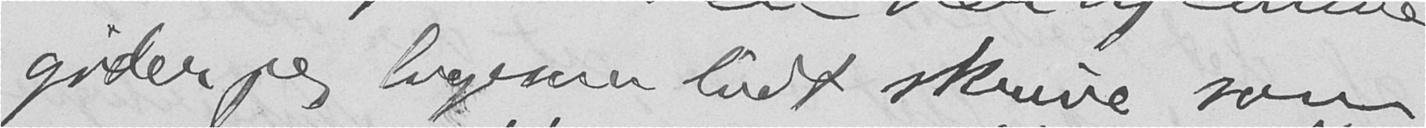gider jeg ligesaa lidt skrive som
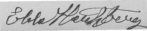Ebbe Hertzberg.
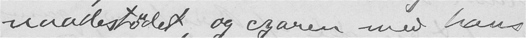naadestødet, og czaren med hans
